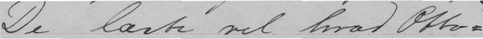- De læste vel hvad Otto-
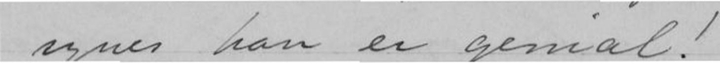synes han er genial!
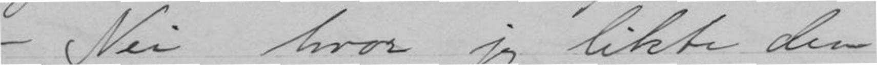- Nei hvor jeg likte den
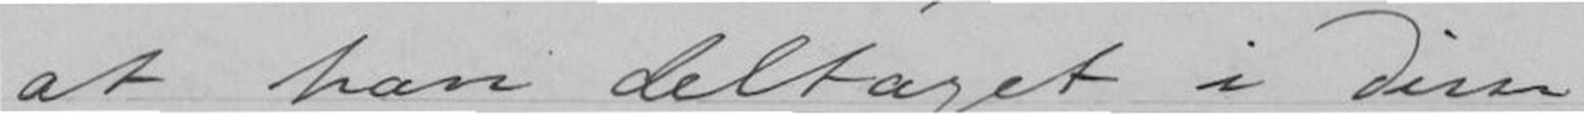at have deltaget i disse
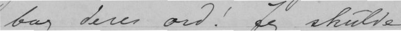bag deres ord! Jeg skulde
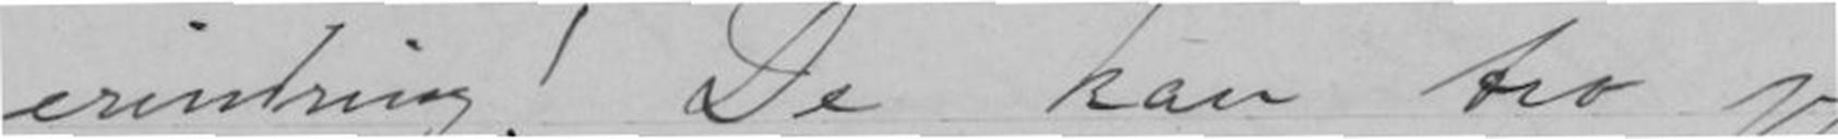erindring! De kan tro jeg
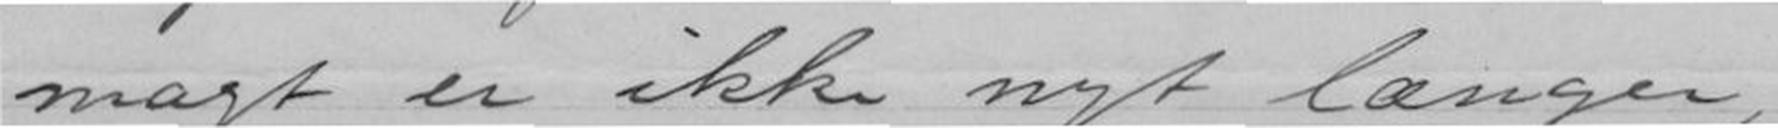magt er ikke nyt længer,
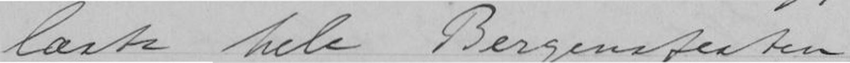læste hele Bergensfesten
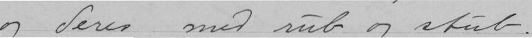og deres med rub og stub!
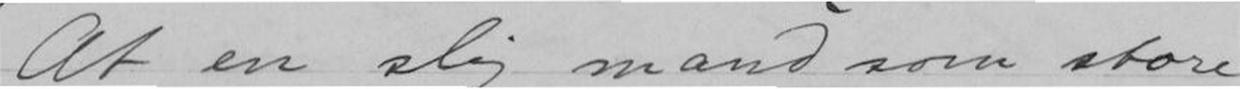At en slig mand som store
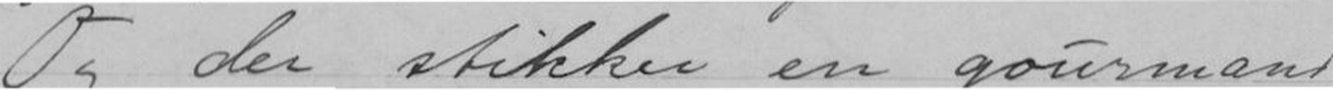Og der stikker en gourmand
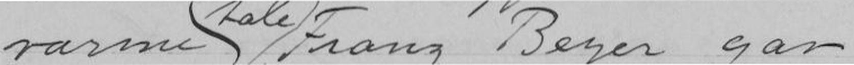varme , Franz Beyer gav
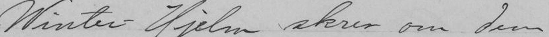Winter-Hjelm skrev om dem
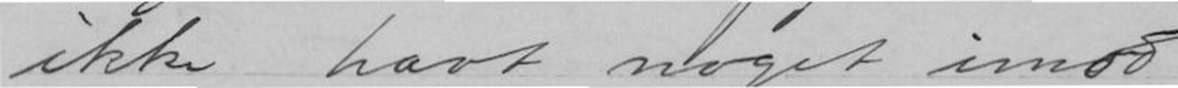ikke havt noget imod
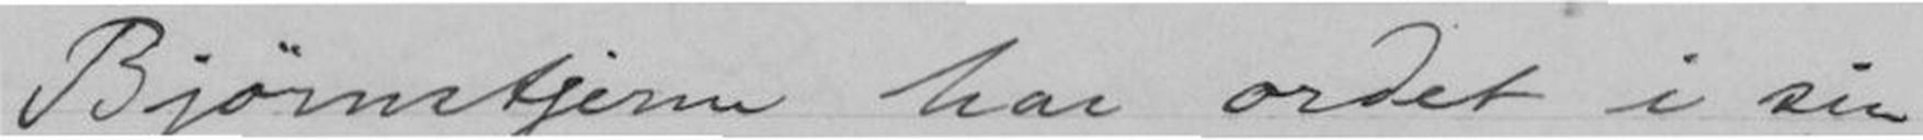Bjørnstjerne har ordet i sin
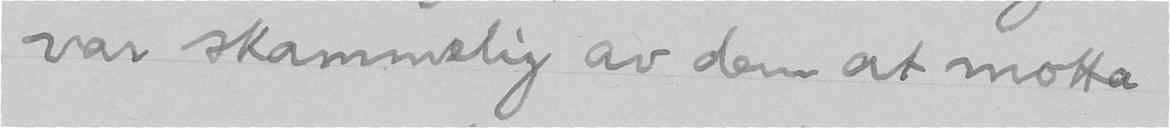var skammelig av dem at motta
